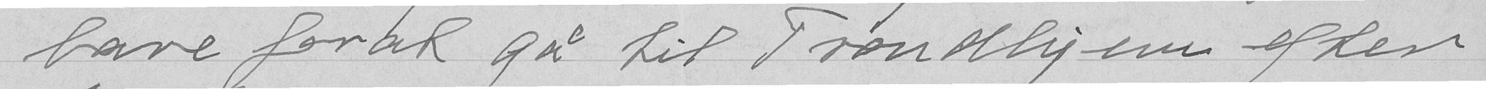bare forat gå til Trondhjem efter
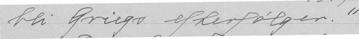bli Griegs efterfølger.
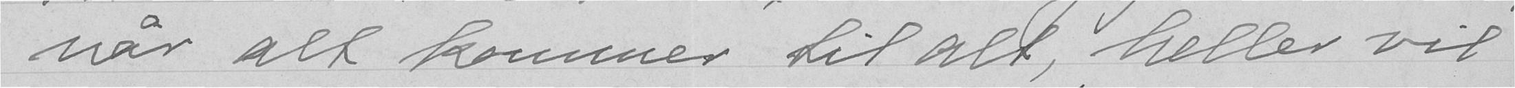når alt kommer til alt, heller vil
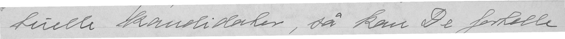tuelle Kandidater, så kan De fortelle
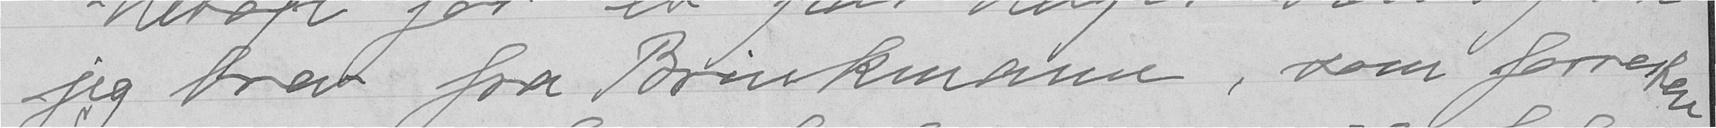jeg brev fra Brinkmann, som forresten
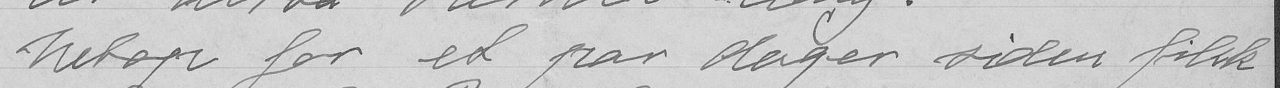Netop for et par dager siden fikk
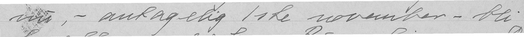nu, - antagelig 1ste november - bli
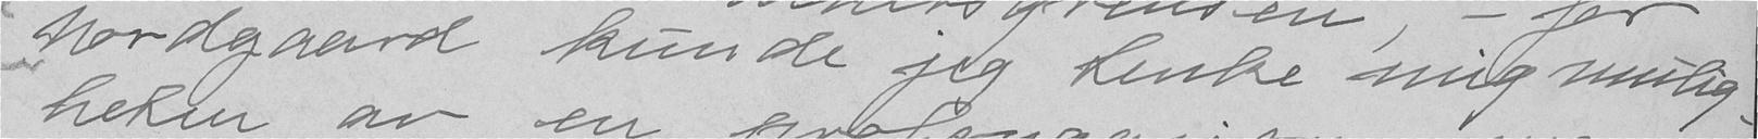Nordgaard kunde jeg tenke mig mulig-
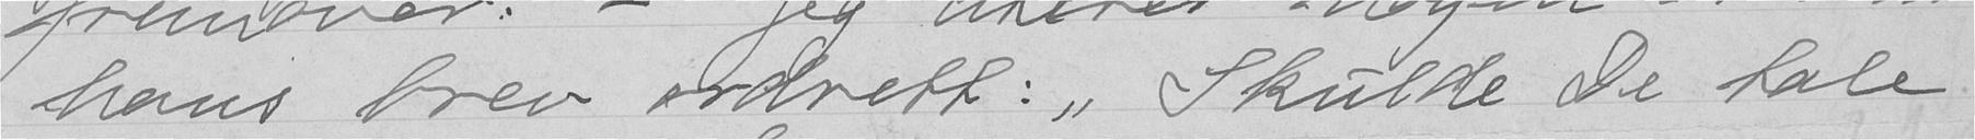hans brev ordrett: Skulde De tale
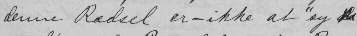denne Rædsel er — ikke at "sy
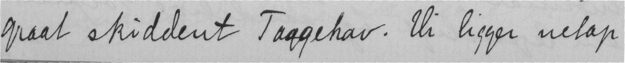graat skiddent Taagehav. Vi ligger netop
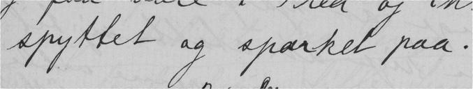spyttet og sparket paa.
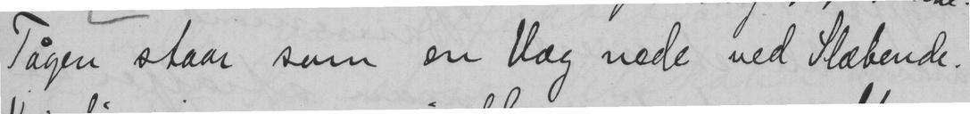Tågen staar som en væg nede ved Slæbende.
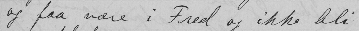og faa være i Fred og ikke bli
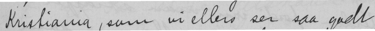Kristiania, som vi ellers ser saa godt
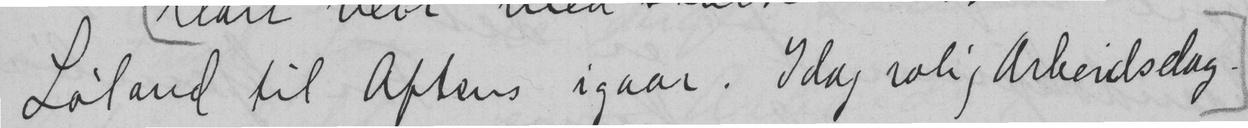Løland til aftens igaar. Idag rolig arbeidsdag.
